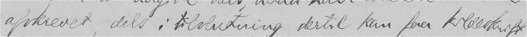afskrevet, dels i tilslutning dertil kan faa kildeskrift-
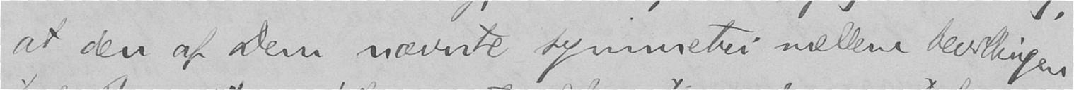at den af Dem nævnte symmetri mellem bevillingen
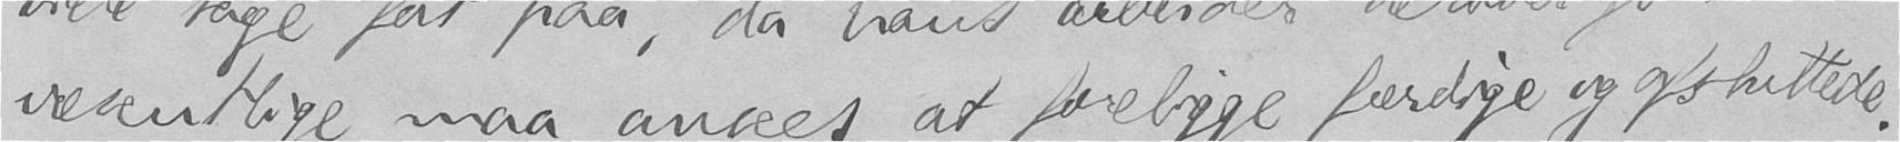væsentlige maa ansees at foreligge færdige og afsluttede.
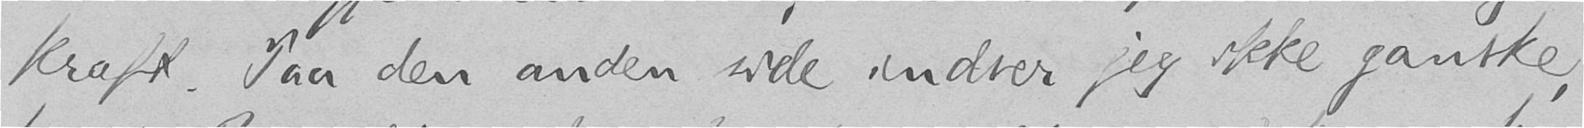kraft. Paa den anden side indser jeg ikke ganske,
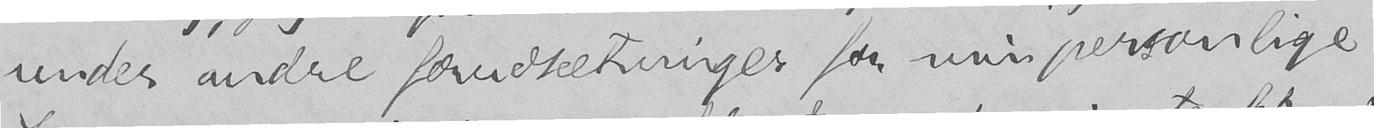under andre forudsætninger for min personlige
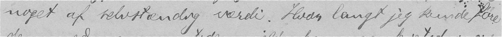noget af selvstændig værdi. Hvor langt jeg kunde føre
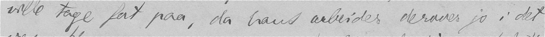ville tage fat paa, da hans arbeider derover jo i det
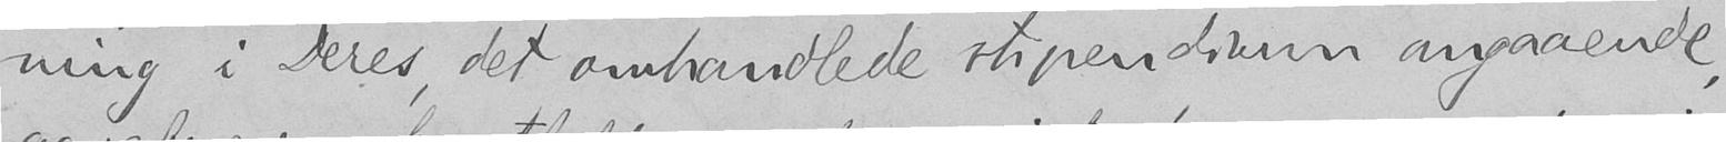ning i Deres, det omhandlede stipendium angaaende,
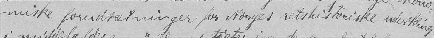miske forudsætninger for Norges retshistoriske udvikling
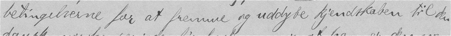betingelserne for at fremme og uddybe kjendskaben til den
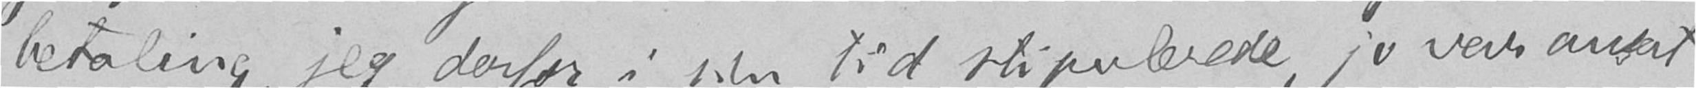betaling, jeg derfor i sin tid stipulerede, jo var ansat
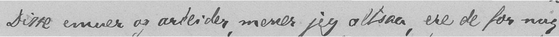Disse emner og arbeider, mener jeg altsaa, ere de for mig
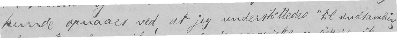kunde opnaaes ved, at jeg understøttedes "til indsamling
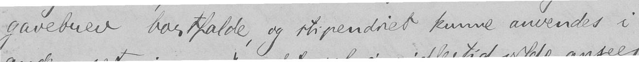gavebrev bortfalde, og stipendiet kunne anvendes i
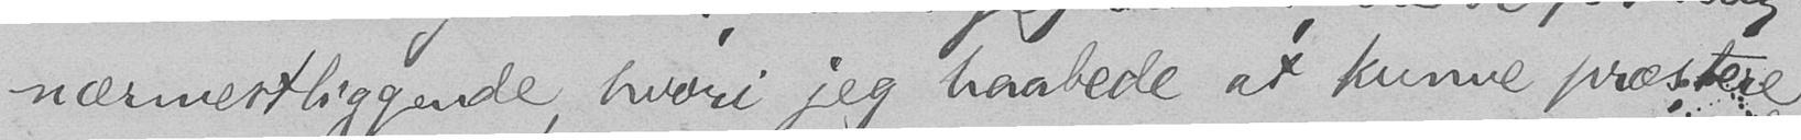nærmestliggende, hvori jeg haabede at kunne præstere
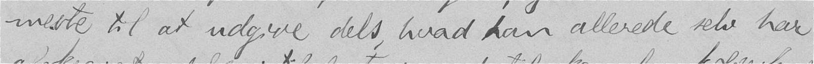meste til at udgive dels, hvad han allerede selv har
